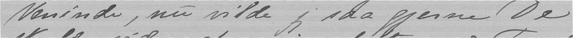Veninde, nu vilde jeg saa gjerne De
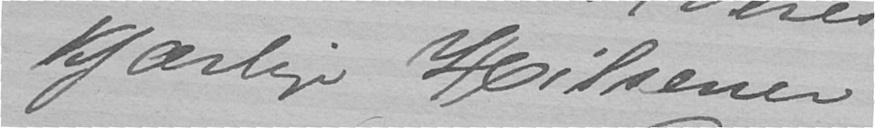kjærlige Hilsener
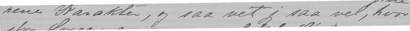rene Karakter, og saa vet jeg saa vel, hvor
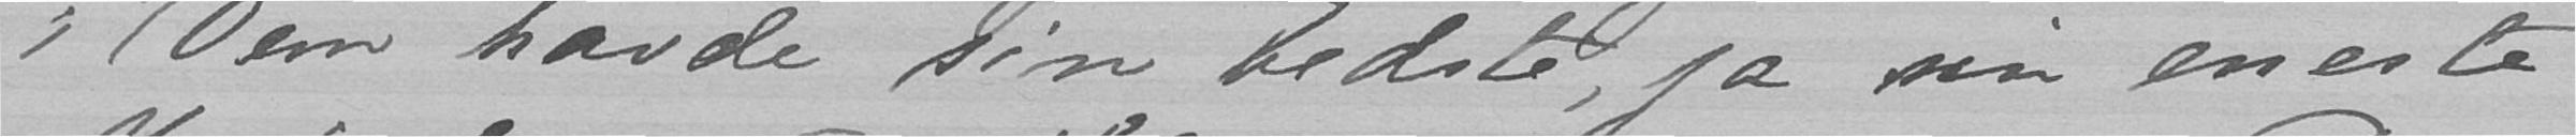i Dem havde sin bedste, ja nu eneste
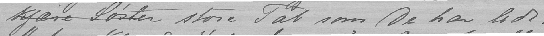kjære Søster store Tab som De har lidt.
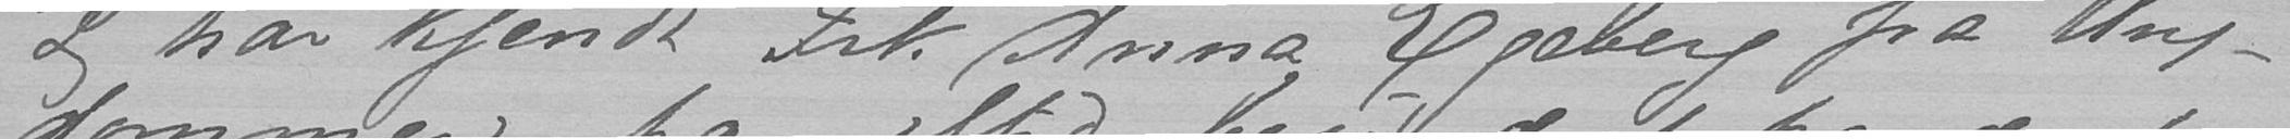Jeg har kjendt Frk Anna Egeberg fra Ung-
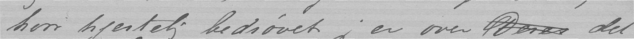hvor hjertelig bedrøvet jeg er over Deres det
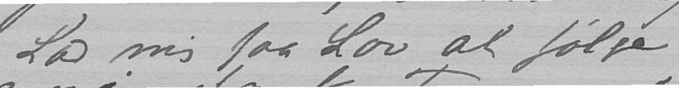Lad mig faa Lov at følge
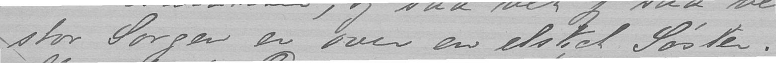stor Sorgen er over en elsket Søster.
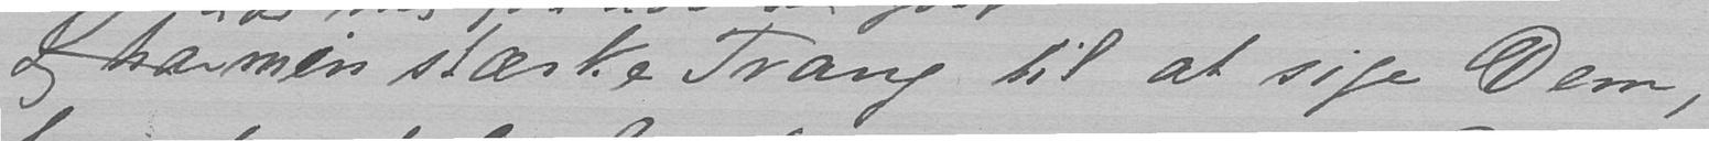Jeg har min stærke Trang til at sige Dem,
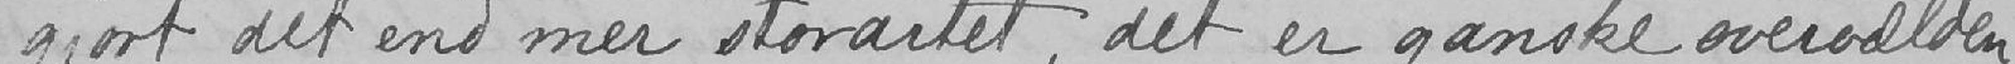gjort det end mer storartet, det er ganske overvælden-
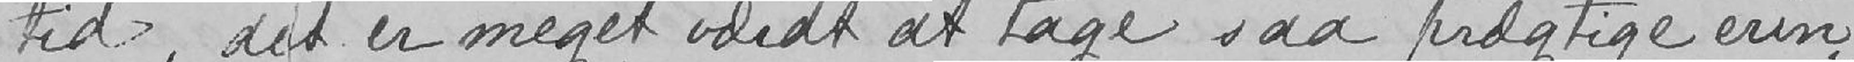tid, det er meget værdt at tage saa prægtige erin-
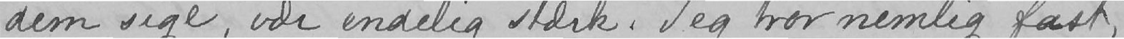dem sige, vær endelig stærk. Jeg tror nemlig fast,
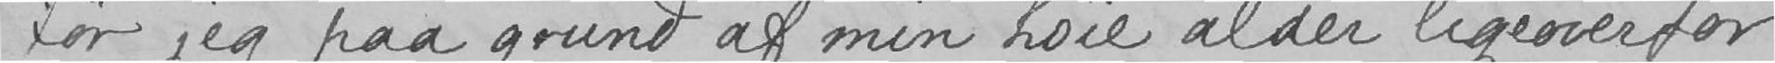tør jeg paa grund af min høie alder ligeoverfor
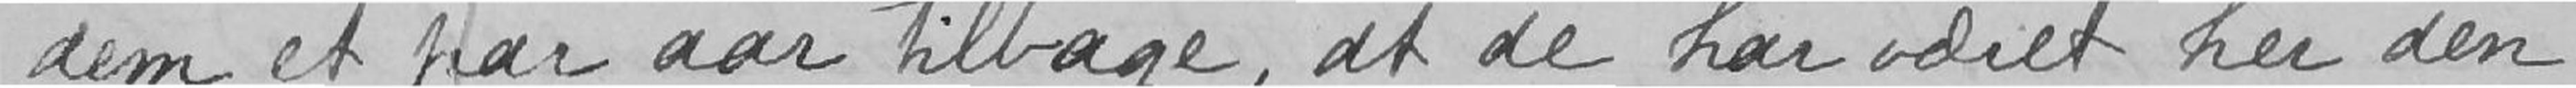dem et par aar tilbage, at de har været her den
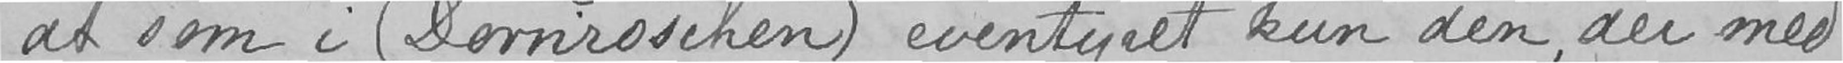at som i («Dornröschen»)? eventyret kun den, der med
